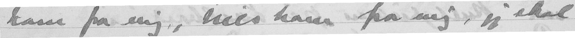ham fra mig, hils ham fra mig, jeg skal
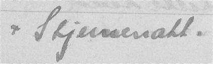Stjernenatt.
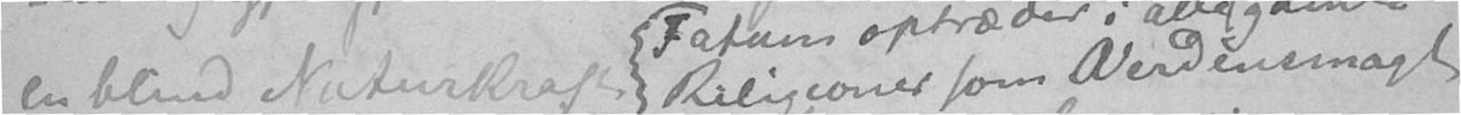en blind Naturkraft. Religionen som Verdensmagt
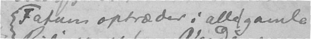Fatum optræder i alle gamle
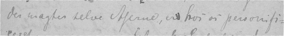der magter selve Athene, er hos os personifi-
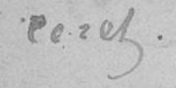ceret.
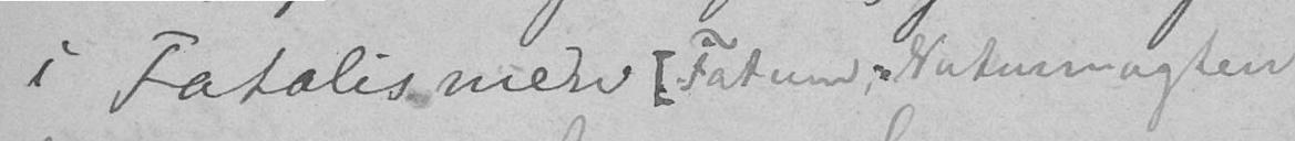i Fatalismen [Fatum, Naturmagten
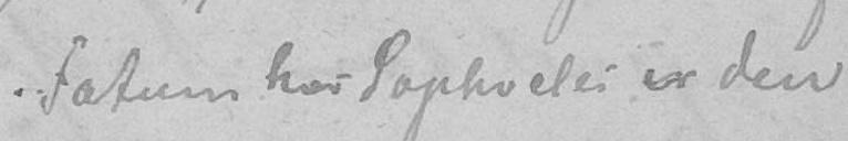Fatum hos Sophocles er den
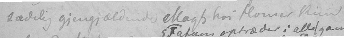sædelig gjengjældende Magt hos Homer kun
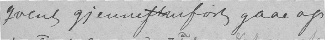qvent gjennemført gaae op
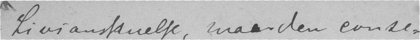Livsanskuelse, maa den conse-
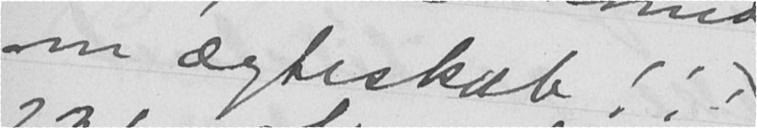om ægteskab!!!
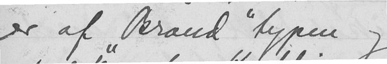er af "Brand" typen og
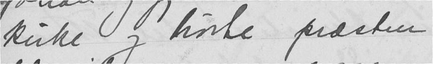kirke og hørte præsten
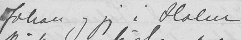Johan og jeg i Holm
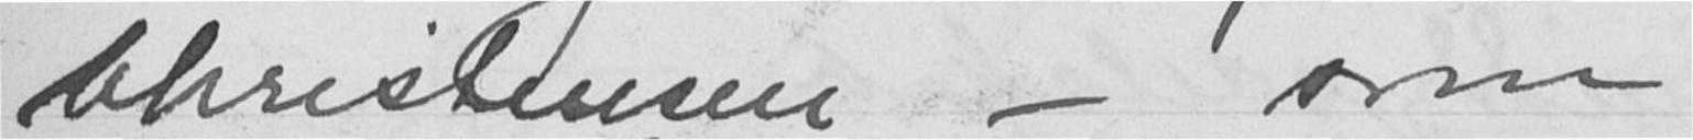Christensen - som
